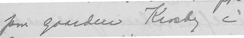paa gaarden Krosby i
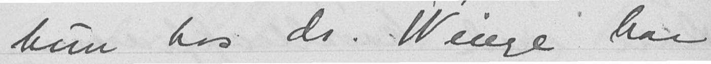hun hos dr. Winge har
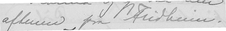aftenen paa Fridheim.
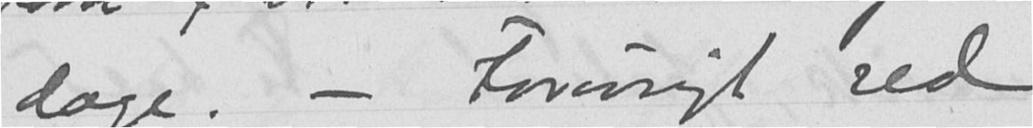dage. - Forøvrigt red
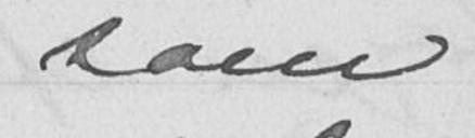som
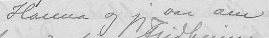Hanna og jeg var om
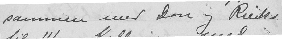sammen med Don og Riecks
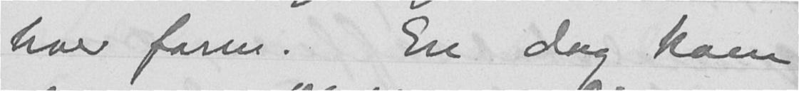hver form. En dag kom
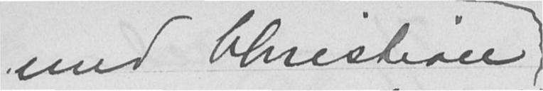med Christian
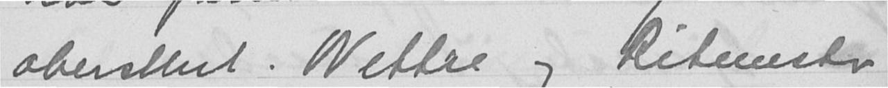oberstlnt. Wettre og Ritmester
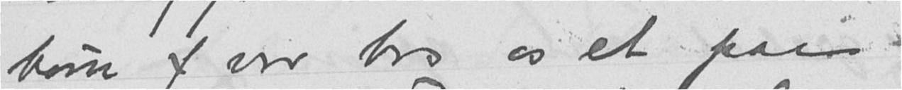børn f var hos os et par
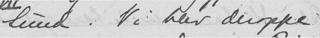Sund. Vi blev deroppe
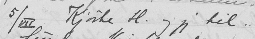5/VII Kjørte H. og jeg til
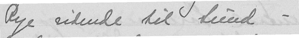Rye ridende til Sund -
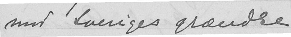mod Sveriges grændse
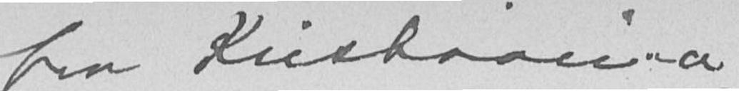fra Kristiania
